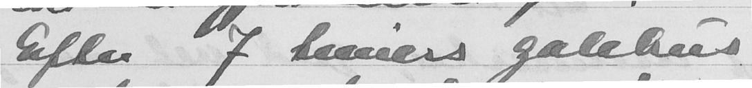Efter 7 timers galehus
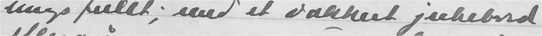unge fullt; med et vakkert julebord
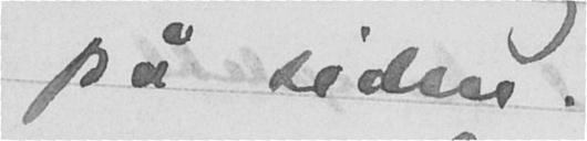på siden.
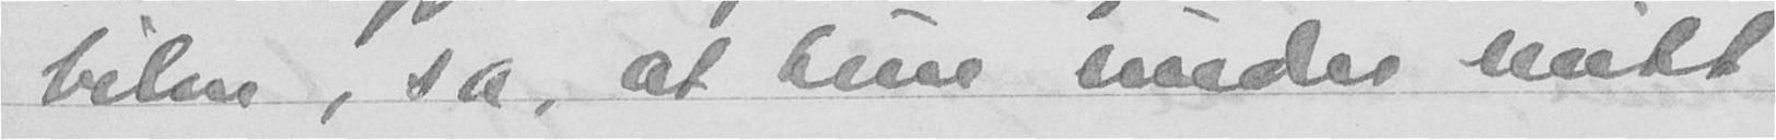bilen, sa, at hen under mitt
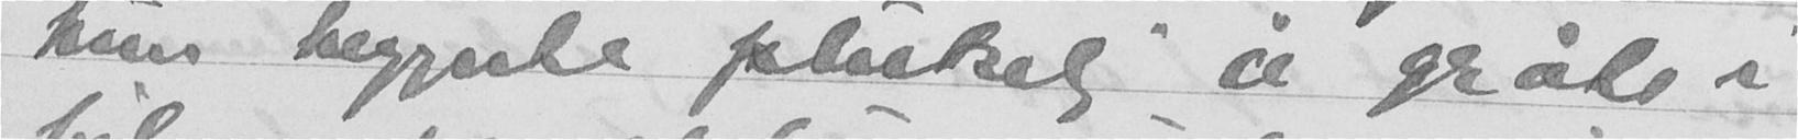hun begynte plutselig å gråte i
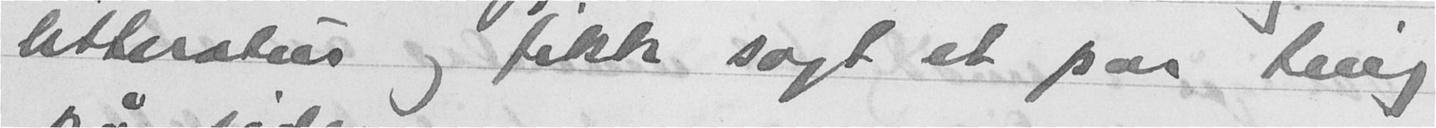litteratur og fikk sagt et par ting
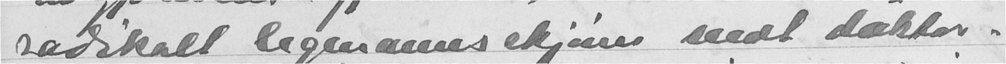radikalt legmannsskjønn mot doktor-
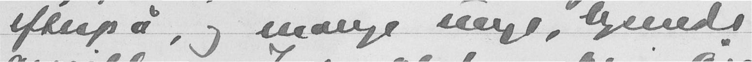efterpå, og mange unge, lysnede
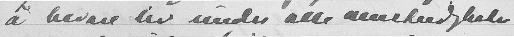å bevare liv under alle omstendigheter
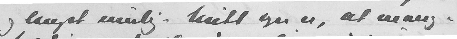og lengst mulig. Mitt syn er, at mang-
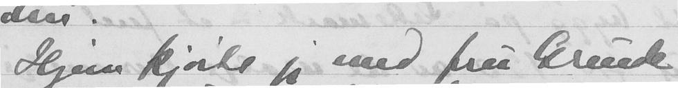Hjem kjørte jeg med fru Gunde
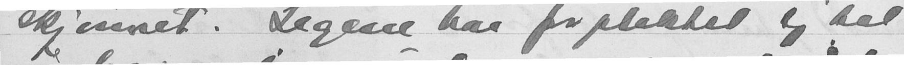skjønnet. Legene har forpliktet sig til
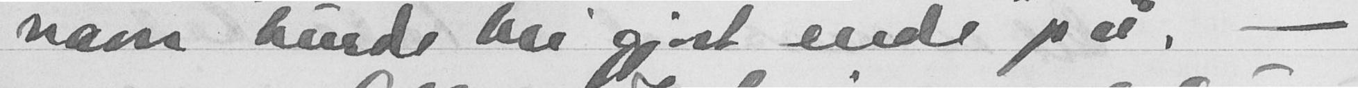navn burde bli gjort ende på. -
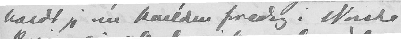holdt jeg om kvelden foredrag i Norske
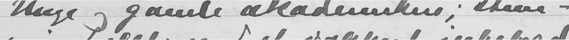Unge og gamle akademikere; store -
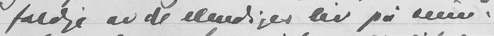foldige av de elendigste liv på sinn-
